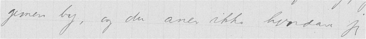gemen by, og du aner ikke hvordan jeg
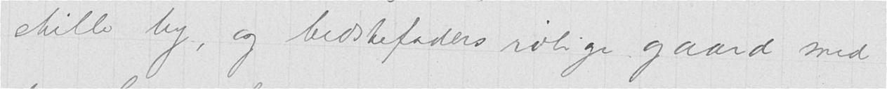stille by, og bedstefaders rolige gaard med
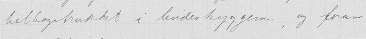tilbagetrukket i lindeskyggerne, og foran
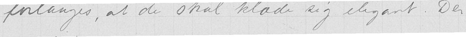forlanges, at de skal klæde sig elegant. Der
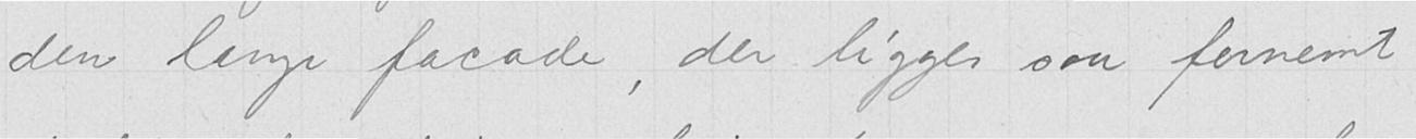den lange facade, der ligger saa fornemt
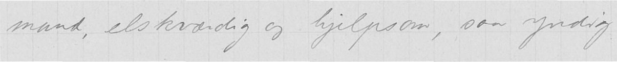mand, elskværdig og hjelpsom, saa yndig
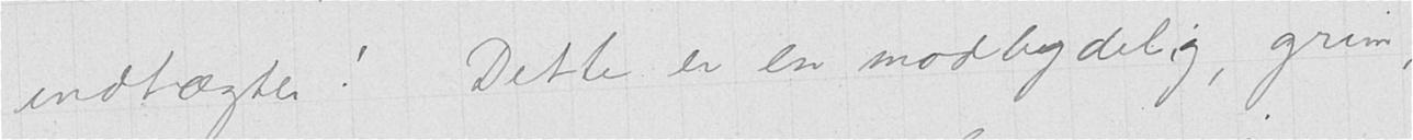indtægte! Dette er en modbydelig, grim,
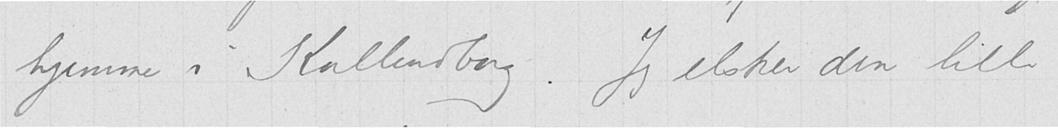hjemme i Kallundborg. Jeg elsker din lille
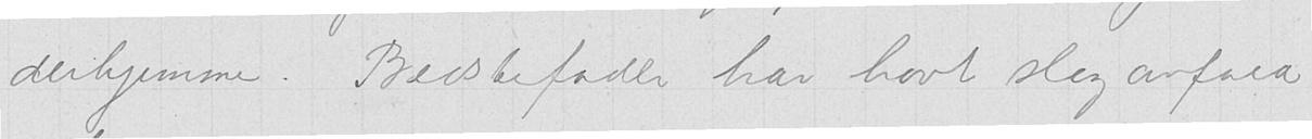derhjemme. Bedstefader har havt slag anfald
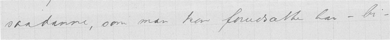saadanne, som man kan forudsætte har - bi-
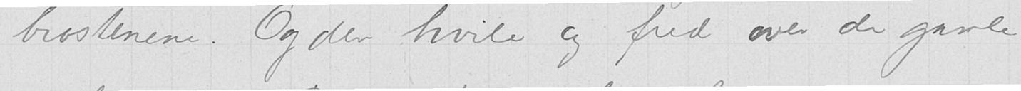brostenene. Og den hvile og fred over de gamle
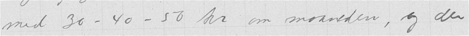med 30 - 40 - 50 kr om maaneden, og der
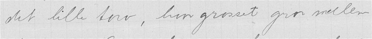det lille torv, hvor gresset gror mellem
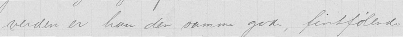verden er han den samme gode, fintfølende
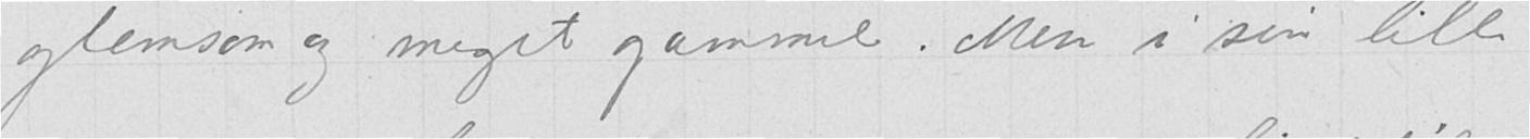glemsom og meget gammel. Men i sin lille
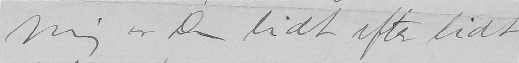mig er Du lidt efter lidt
